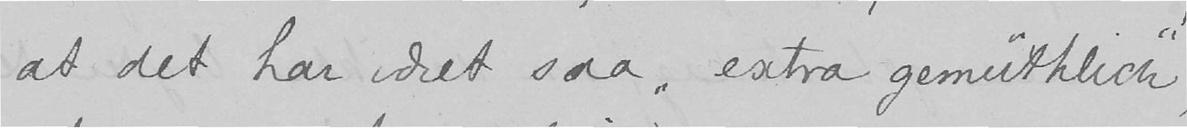at det har været saa «extra gemüthlich»,
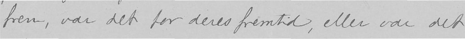frem, var det for deres fremtid, eller var det
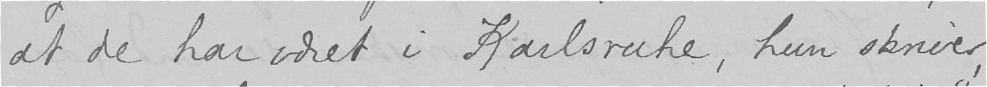at de har været i Karlsruhe, hun skriver,
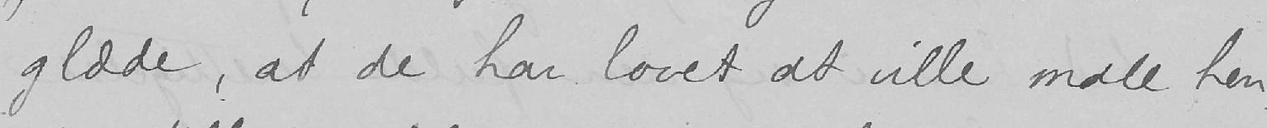glæde, at de har lovet at ville male hen-
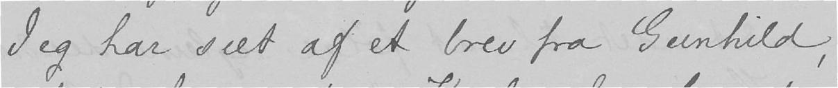Jeg har seet af et brev fra Gunhild,
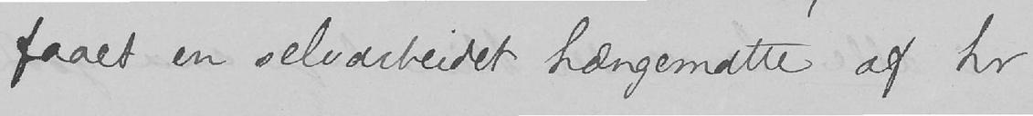faaet en selvarbeidet hængematte af hr
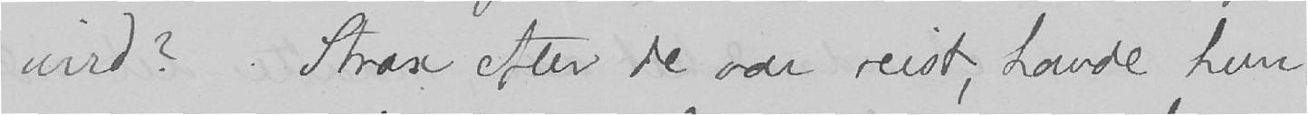wird»? Strax efter de var reist, havde hun
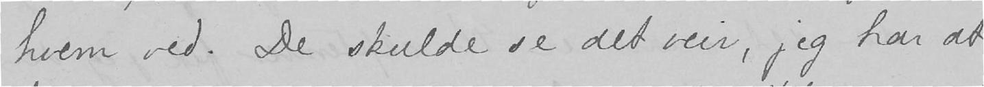hvem ved. De skulde se det veir, jeg har at
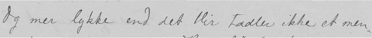Og mer lykke end det blir taaler ikke et men
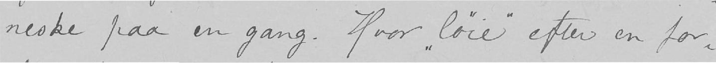neske paa en gang. Hvor «løie» efter en for-
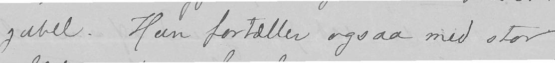jubel. Hun fortæller ogsaa med stor
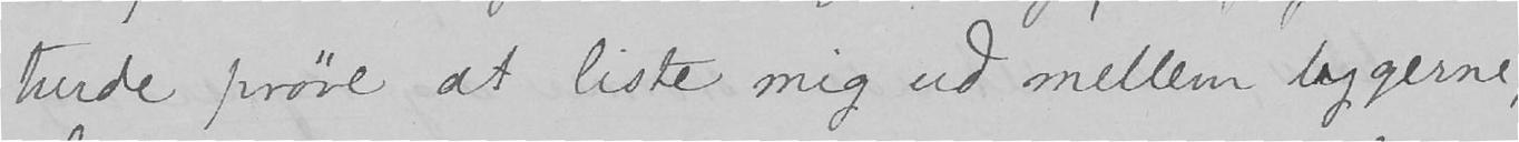turde prøve at liste mig ud mellem bygerne,
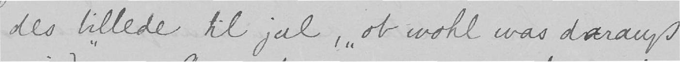des billede til jul, «ob wohl was darauß
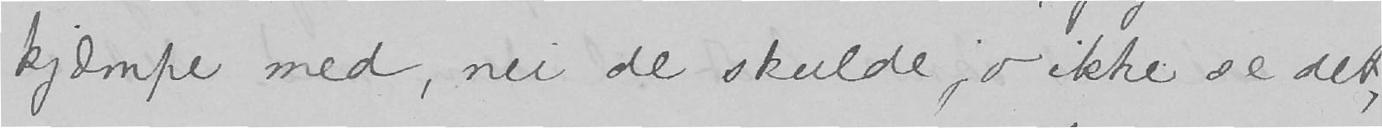kjæmpe med, nei de skulde jo ikke se det,
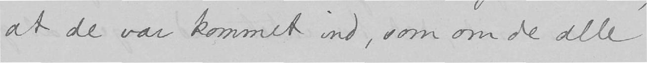at de var kommet ind, som om de alle
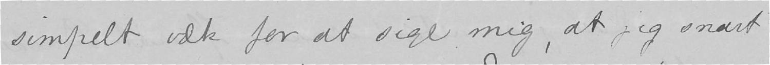simpelt væk for at sige mig, at jeg snart
